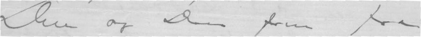Dem og Deres frue fra
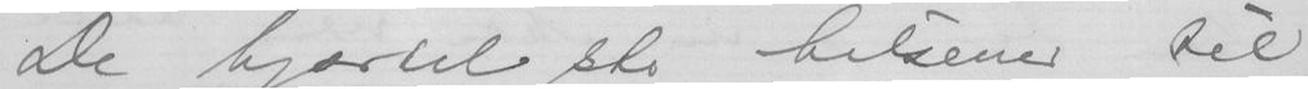De hjærteligste hilsener til
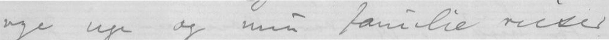rige uge og min familie reiser
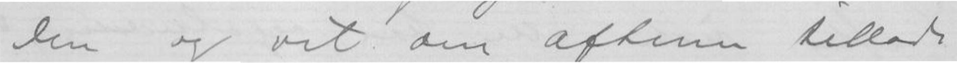den og vet om aftenen tillade
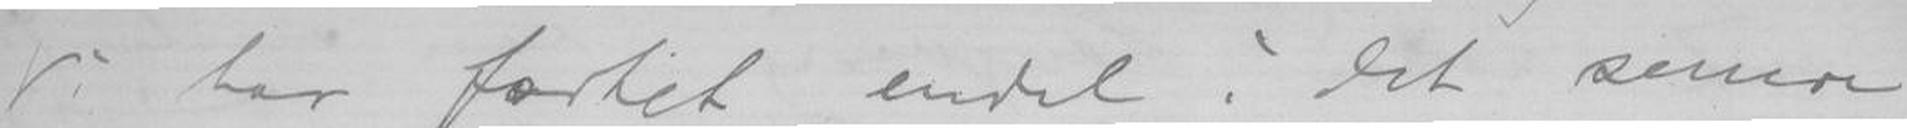Vi har fartet endel i det senere
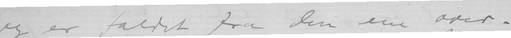og er faldet fra den ene over-
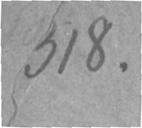318.
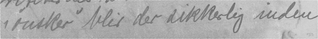ønsker blir der sikkerlig inden
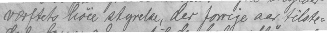værftets høie styrelse, der forrige aar tilste-
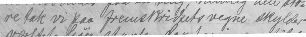re tak vi paa fremskridtets vegne skylder
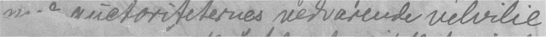med auctoriteternes vedvarende velvilie
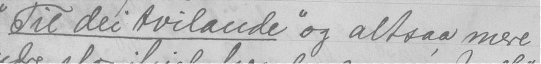"Til dei tvilande" og altsaa mere
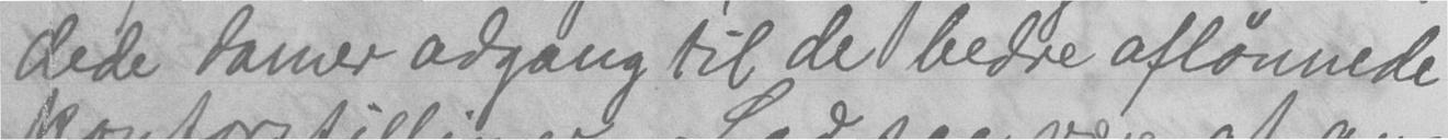dede damer adgang til de bedre aflønnede
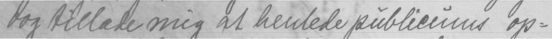dog tillade mig at henlede publicims op-
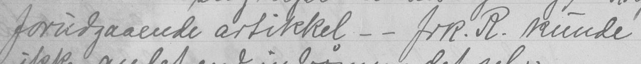forudgaaende artikkel - - frk. R. kunde
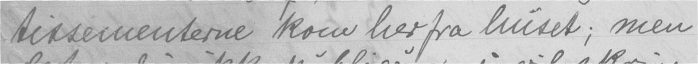tissementerne kom her fra huset; men
